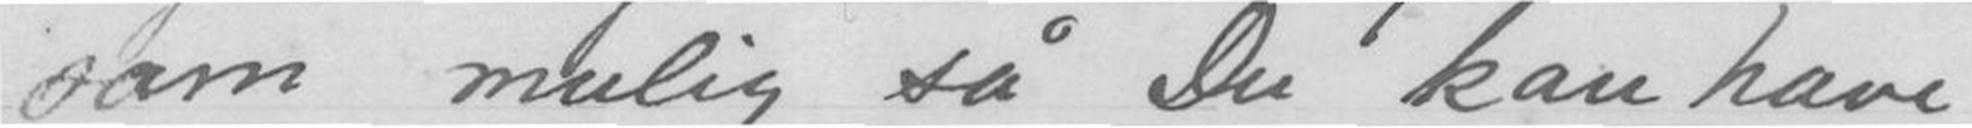som mulig så Du kan have
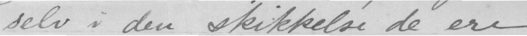selv i den skikkelse de ere
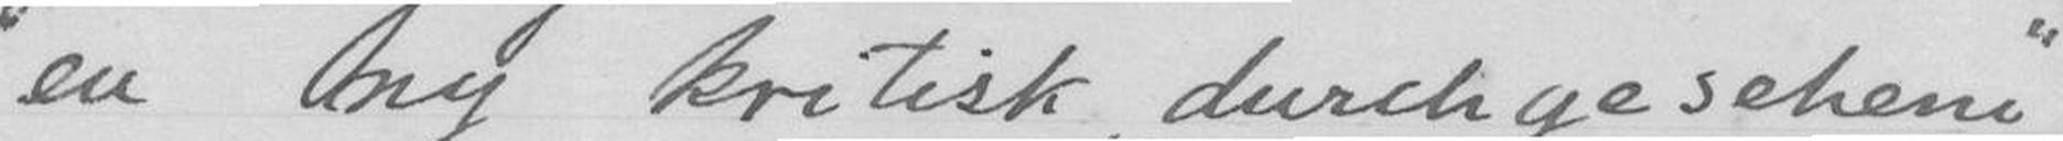en ny kritisk "durchgesehene"
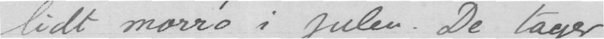lidt morro i julen. De tager
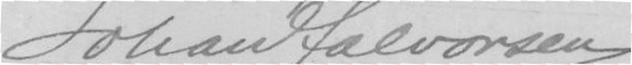Johan Halvorsen
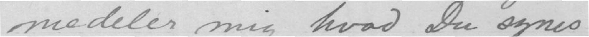medeler mig hvad Du synes
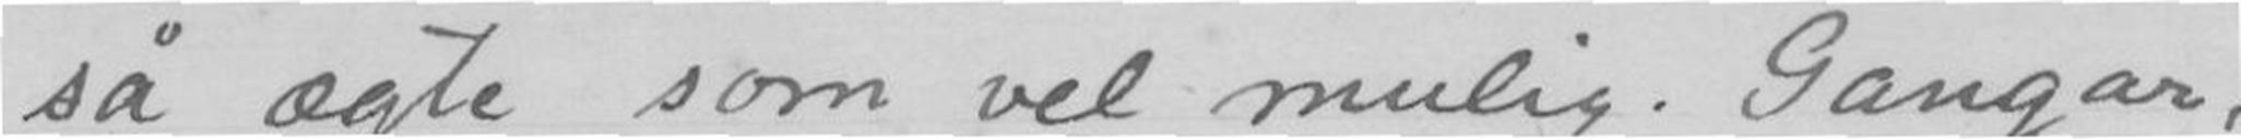så ægte som vel mulig. Gangar,
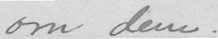om dem.
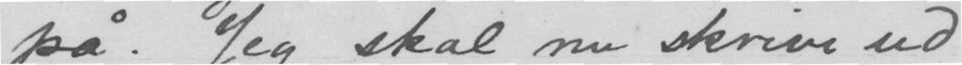på. Jeg skal nu skrive ud
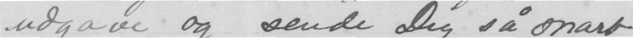udgave og sende Deg så snart
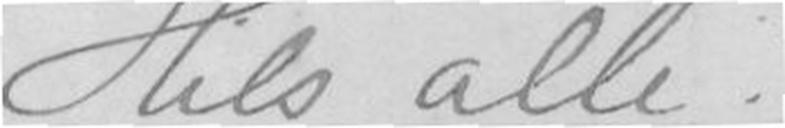Hils alle!
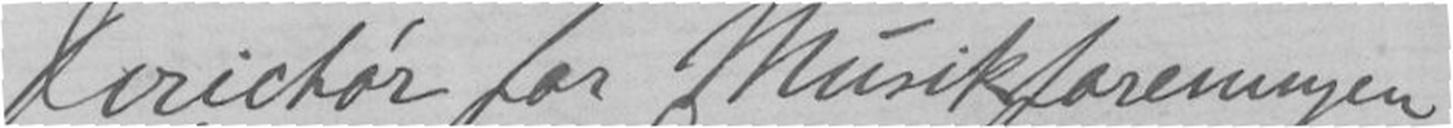Dirictør for musikforeningen
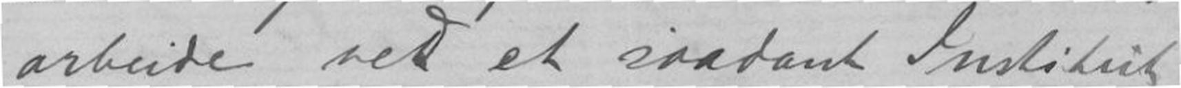arbeide ved et saadant Institut
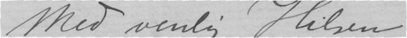Men venlig Hilsen
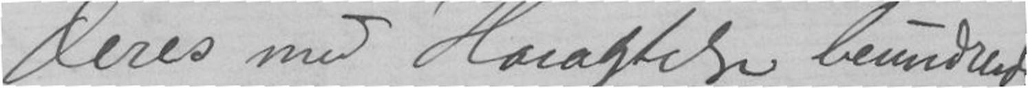Deres med høiagtelse beundrelse
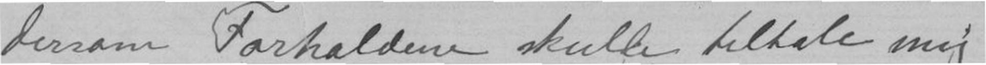dersom Forholdene skulle tillade mig
